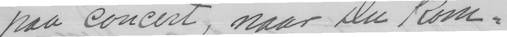paa concert, naar Du kom-
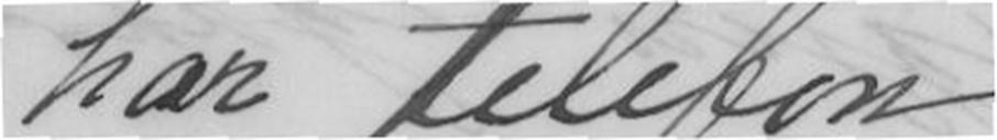har telefon
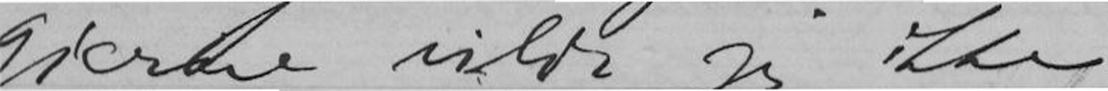gjerne vilde jeg ikke
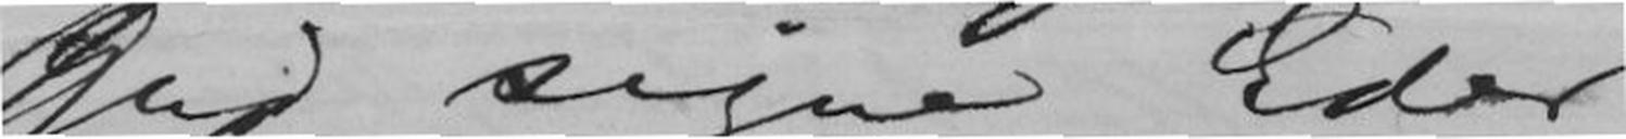Gud signe Eder
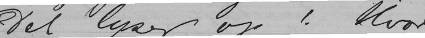Det lyser op! Hvor
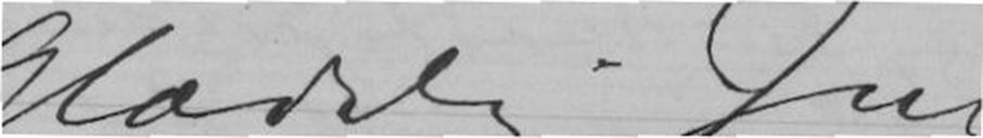Glædelig Jul!
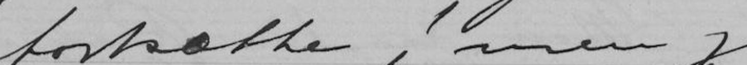fortsætte, men jeg
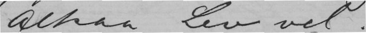Altsaa Lev vel!
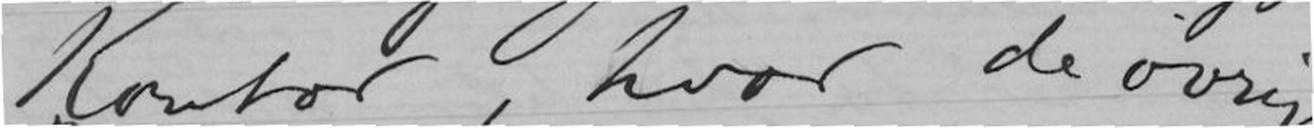Kontor, hvor de øvrige
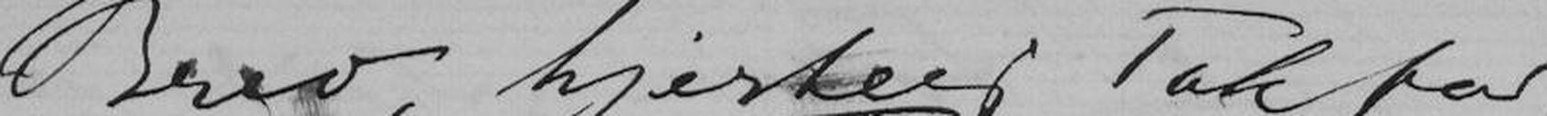Brev, hjertlig Tak for
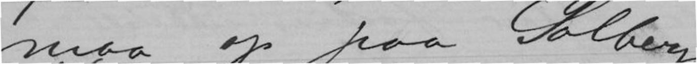maa op paa Solbergs
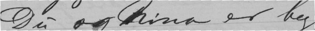Du og Nina er begge
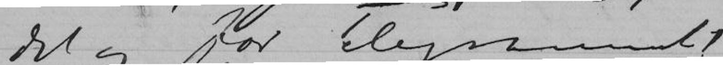det og for Telegrammet!
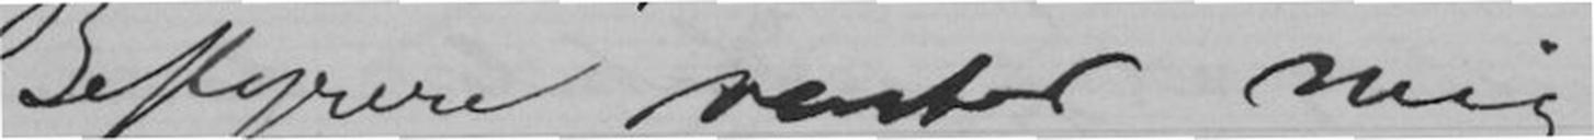Bestyrere venter mig.
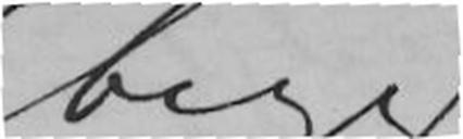begge!
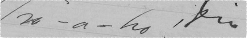Tra-a-ho! Din
